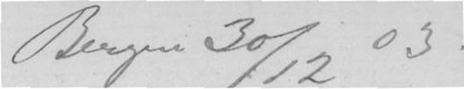Bergen 30/12 03.
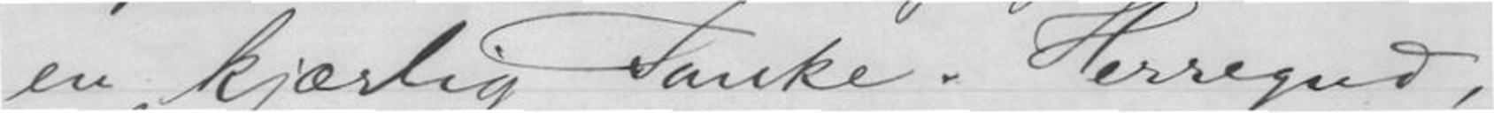en kjærlig Tanke. Herregud,
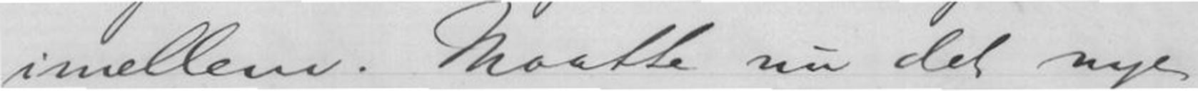imellem. Maatte nu det nye
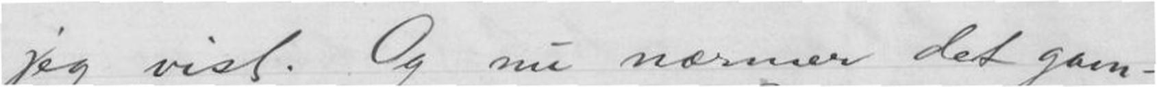jeg vist. Og nu nærmer det gam-
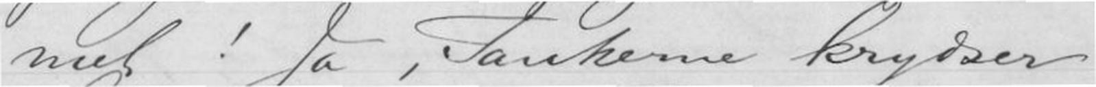met! Ja, Tankerne krydser
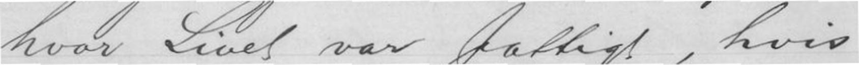hvor Livet var fattigt, hvis
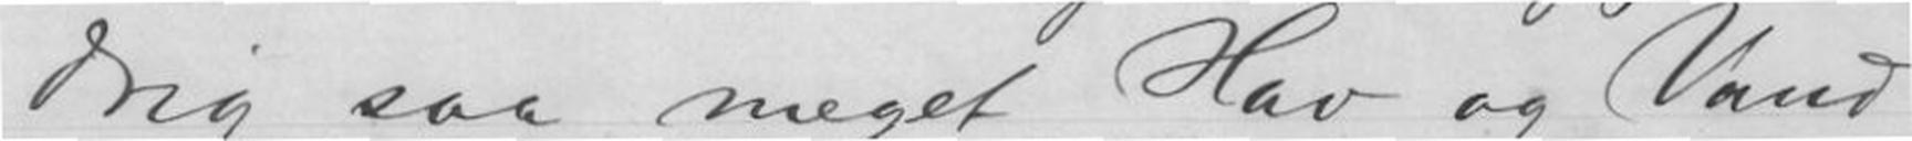drig saa meget Hav og Vand
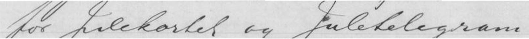for Julekortet og Juletelegram-
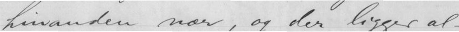hinanden nær, og der ligger al-
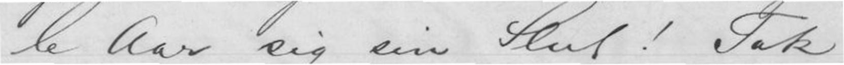le Aar sig sin Slut! Tak
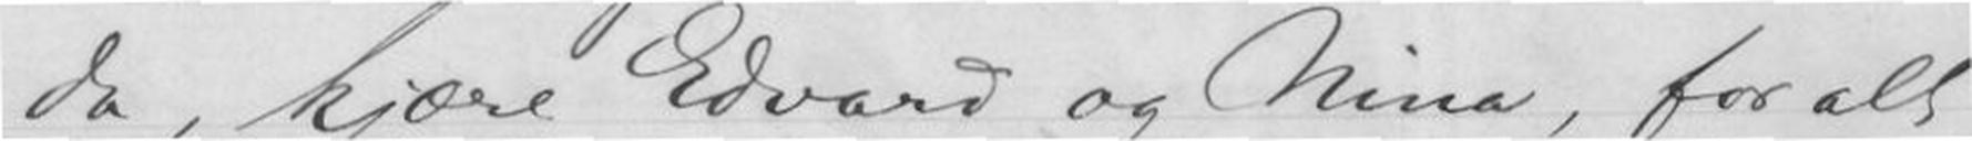da, kjære Edvard og Nina, for alt
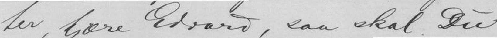ter, kjære Edvard, saa skal Du
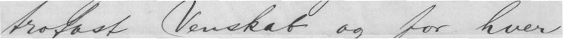trofast Venskab og for hver
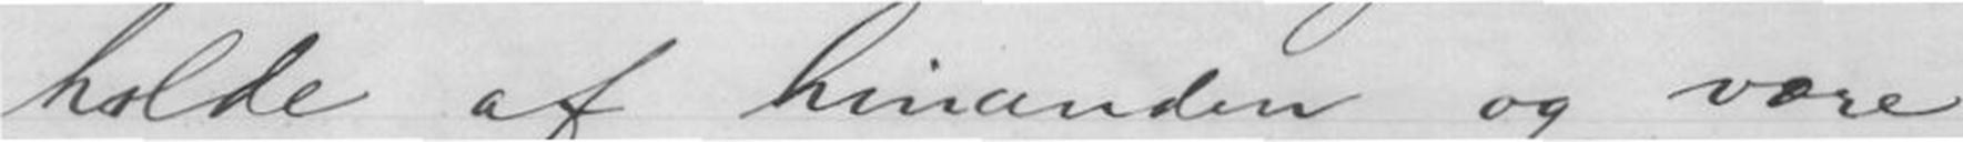holde af hinanden og være
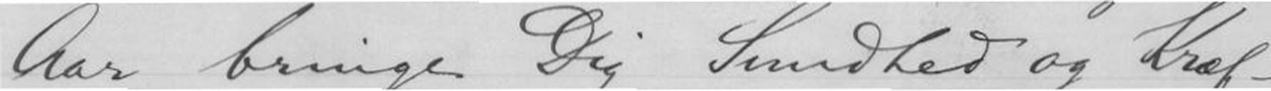Aar bringe Dig Sundhed og Kræf-
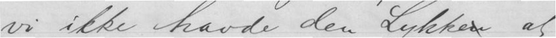vi ikke havde den Lykken at
